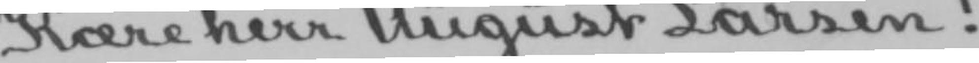Kære herr August Larsen!
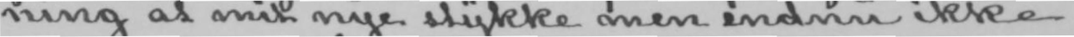ning at mit nye stykke men endnu ikke
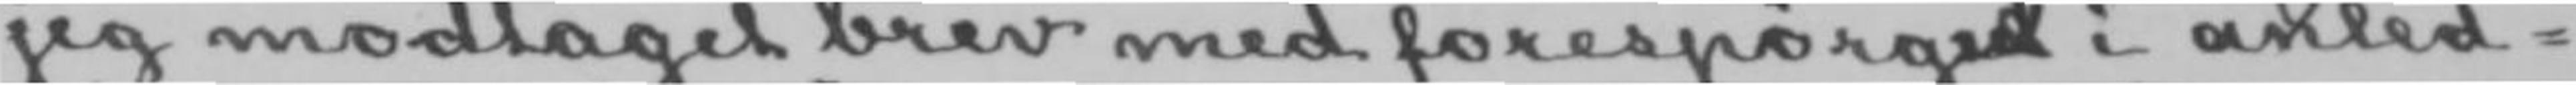jeg modtaget brev med forespørgsel i anled-
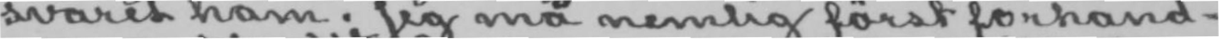svaret ham. Jeg må nemlig først forhand-
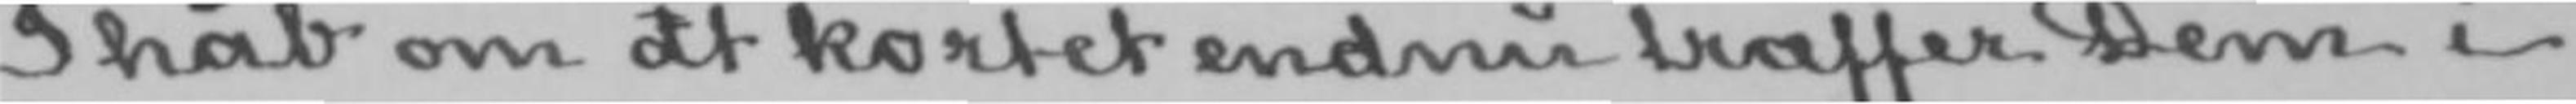I håb om dat kortet endnu træffer Dem i
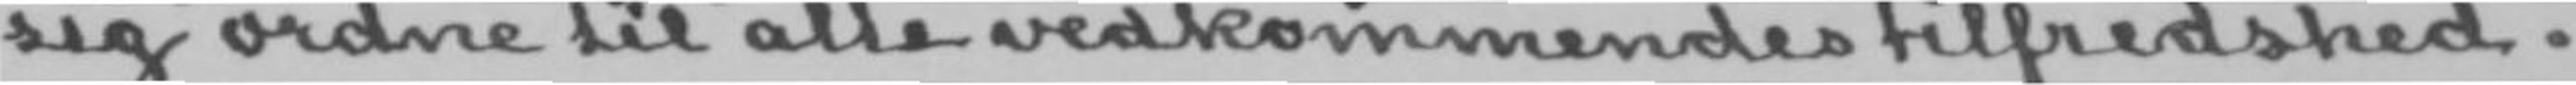sig ordne til alle vedkommendes tilfredshed.
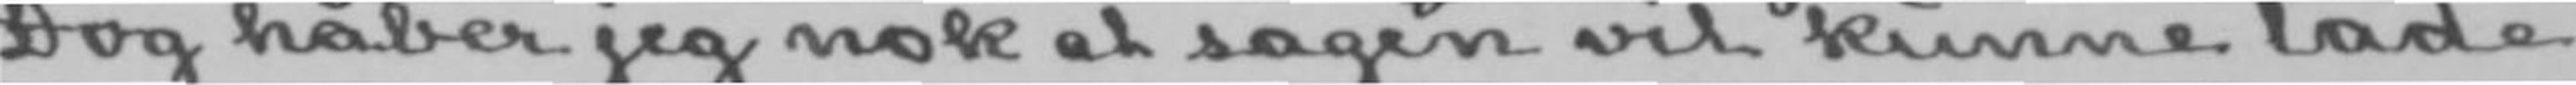Dog håber jeg nok at sagen vil kunne lade
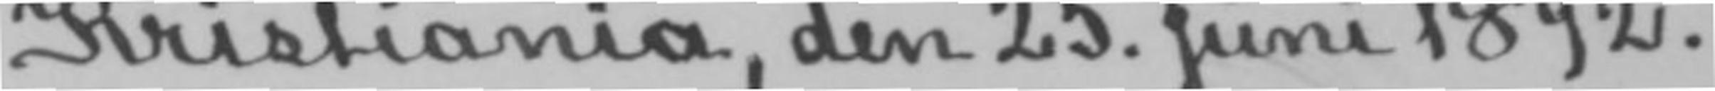Kristiania, den 25. Juni 1892.
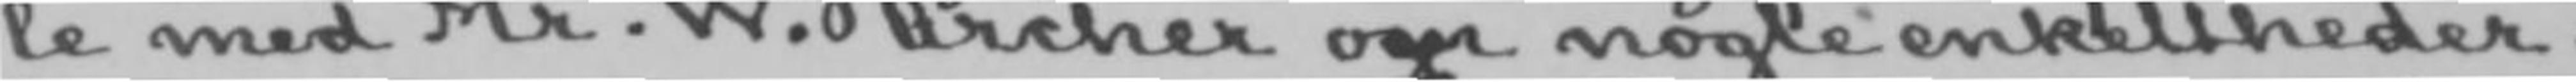le med Mr. W. Archer om nogle enkeltheder.
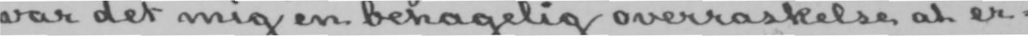var det mig en behagelig overraskelse at er-
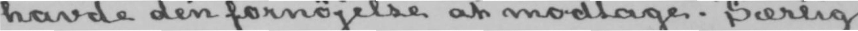havde den fornøjelse at modtage. Særlig
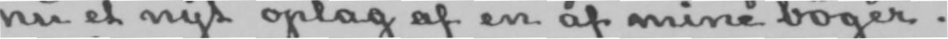nu et nyt oplag af en af mine bøger.
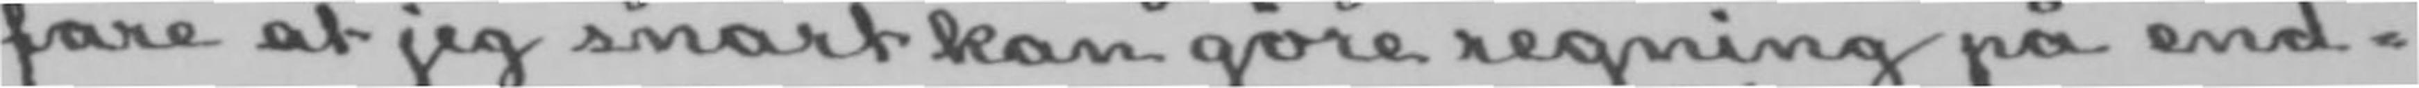fare at jeg snart kan gøre regning på end-
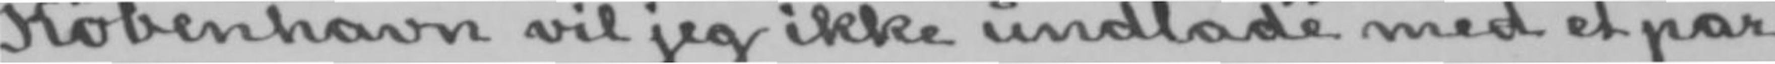København vil jeg ikke undlade med et par
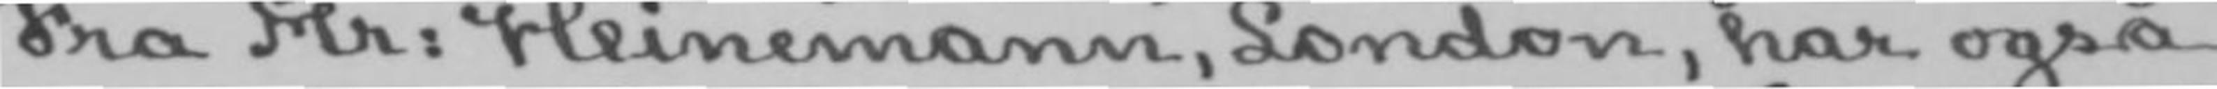Fra Mr: Heinemann, London, har også
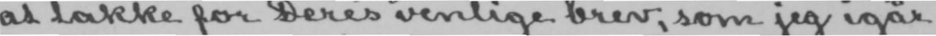at takke for Deres venlige brev, som jeg igår
